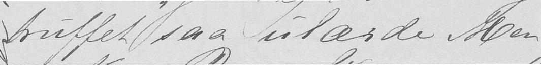truffet saa ulærde Men-
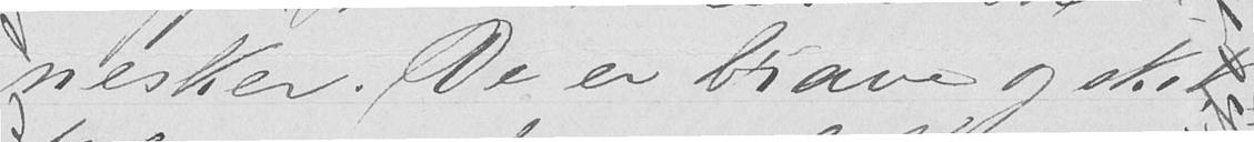nesker. De er brave og skik-
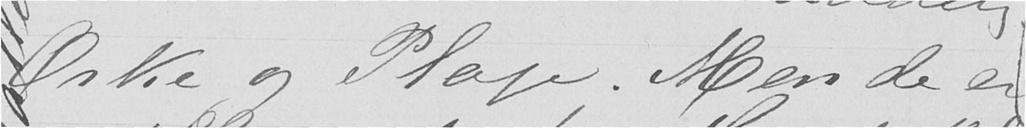Ørke og Plage. Men de er
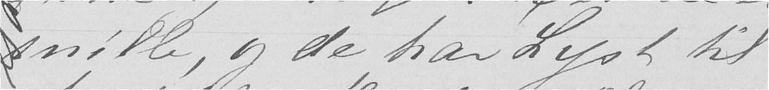Snille, og de har Lyst til
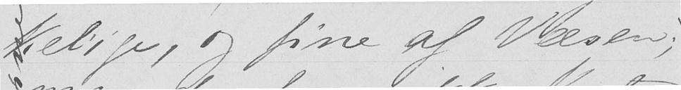kelige, og fine af Væsen,
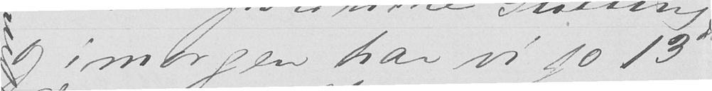og i morgen har vi jo 13de
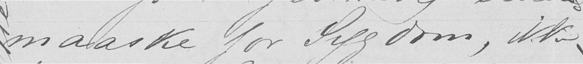maaske for Sygdom, ikke
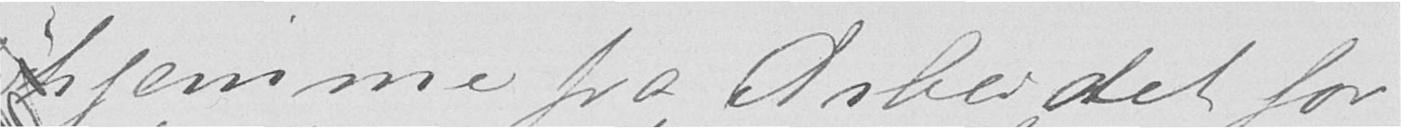hjemme fra Arbeidet for
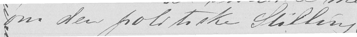om den politiske Stilling,
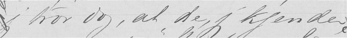jeg tror dog, at de, jeg kjender,
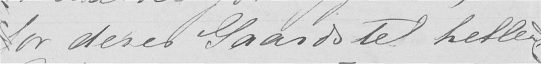for deres Gaardstel heller.
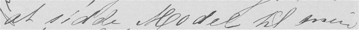at sidde Model til min
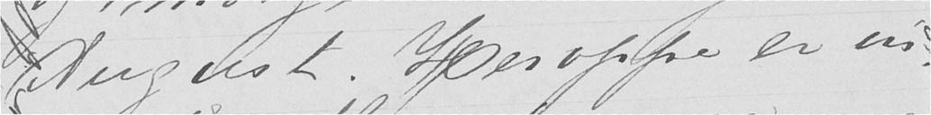August. Her oppe er in-
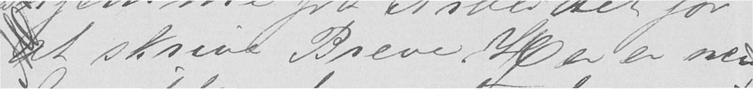et skrive Breve. Her er nem-
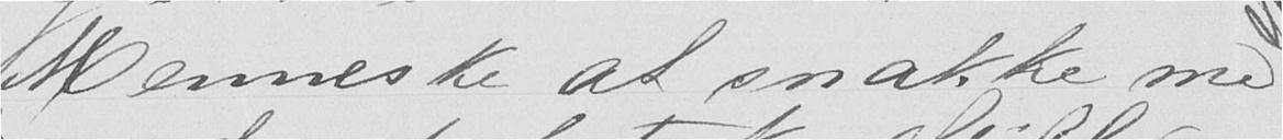Menneske at snakke med
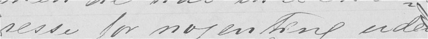resse for nogenting uden
